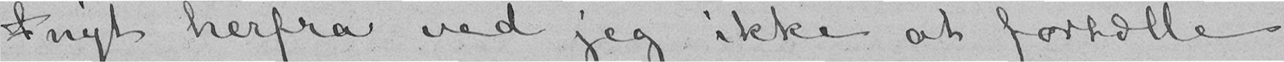nyt herfra ved jeg ikke at fortælle
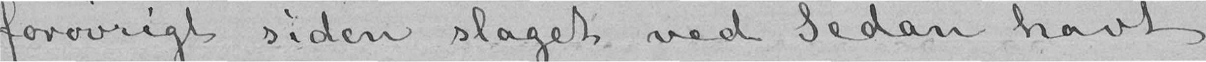forøvrigt siden slaget ved Sedan havt
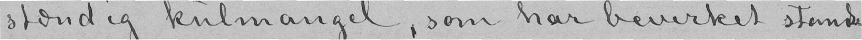stændig kulmangel, som har bevirket stands-
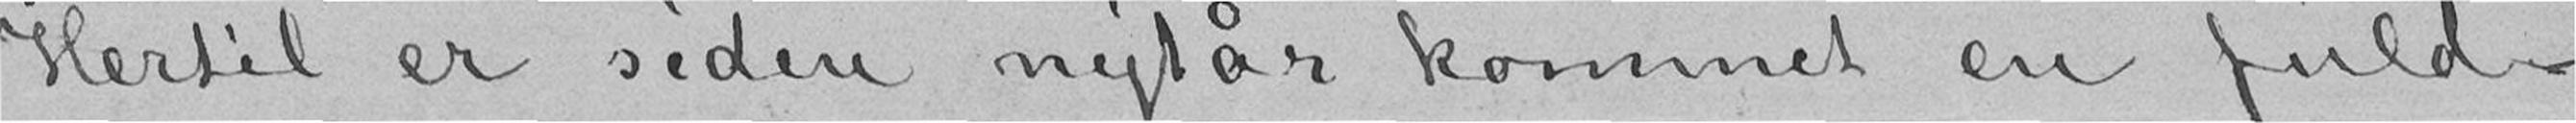Hertil er siden nytår kommet en fuld-
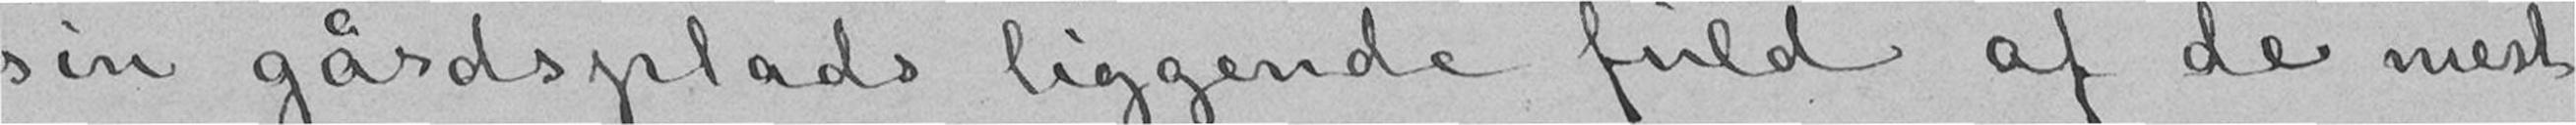sin gårdsplads liggende fuld af de mest
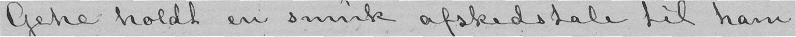Gehe holdt en smuk afskedstale til ham,
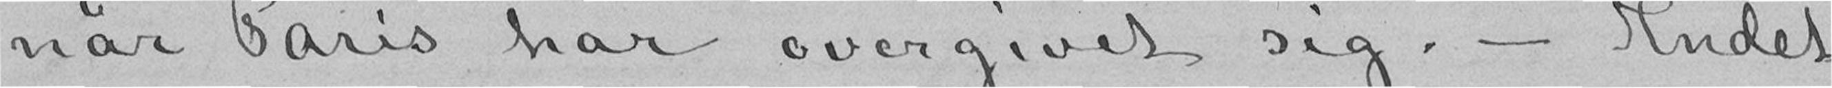når Paris har overgivet sig.- Andet
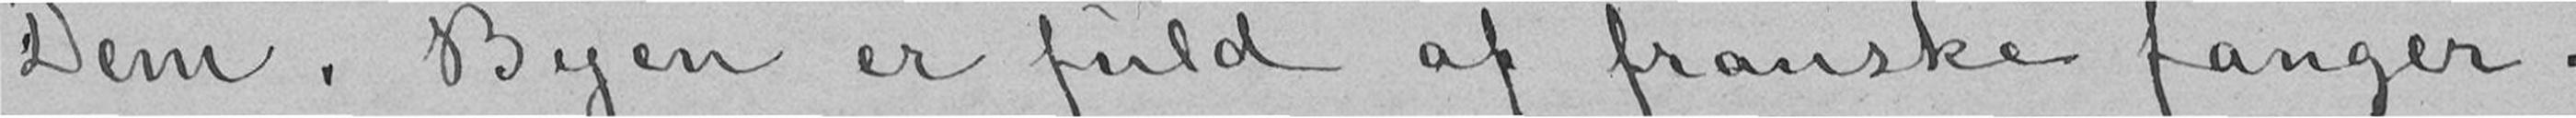Dem. Byen er fuld af franske fanger.
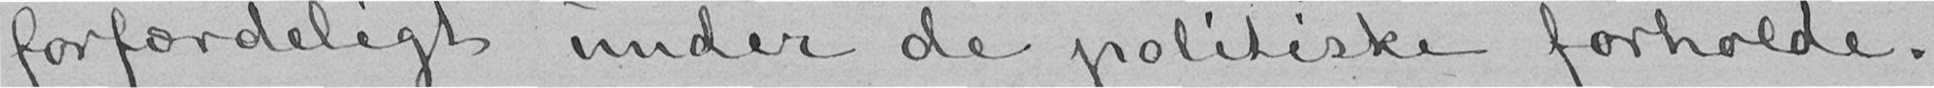forfærdeligt under de politiske forholde.
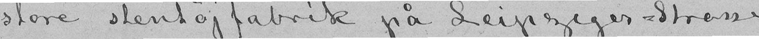store stentøjfabrik på Leipziger-Strasse
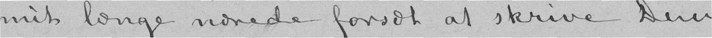mit længe nærede forsæt at skrive Dem
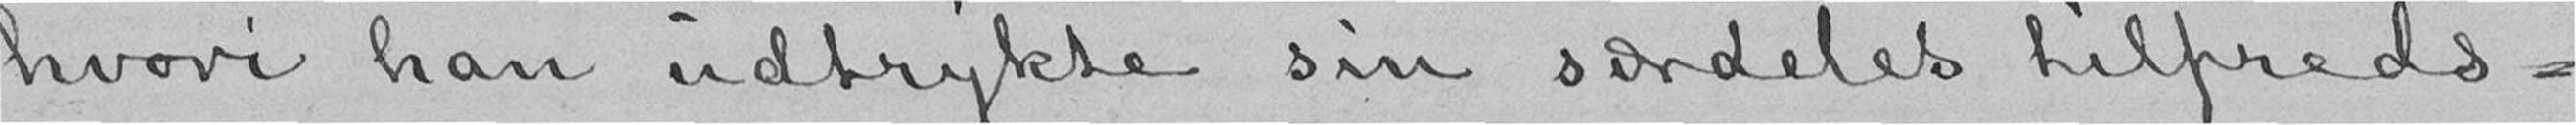hvori han udtrykte sin særdeles tilfreds-
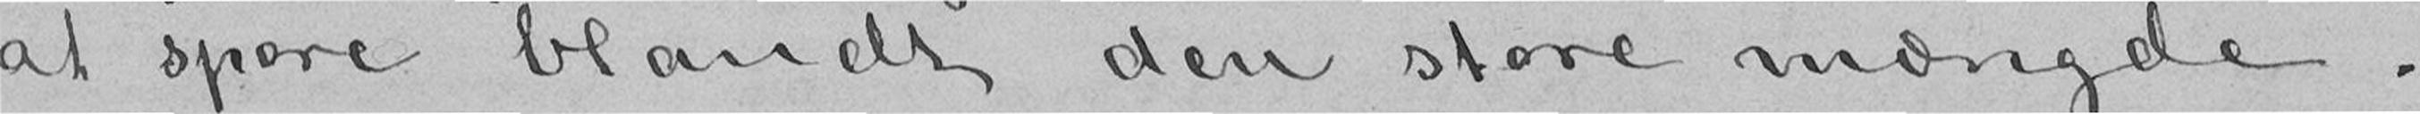at spore blandt den store mængde.
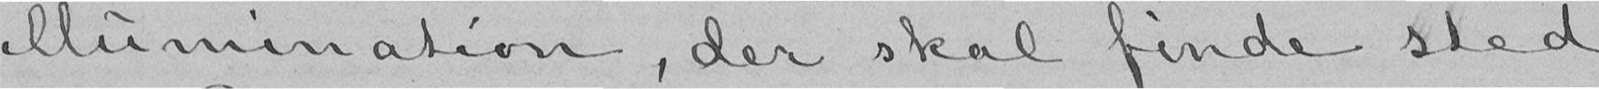illumination, der skal finde sted
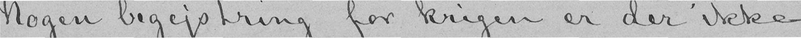Nogen begejstring for krigen er der ikke
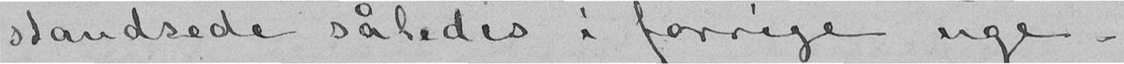standsede således i forrige uge.
